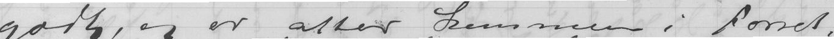godt, og er atter kommen i Forret
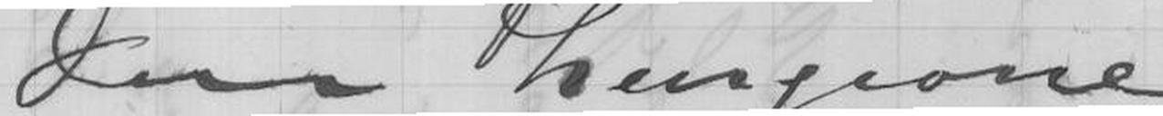Din hengivne
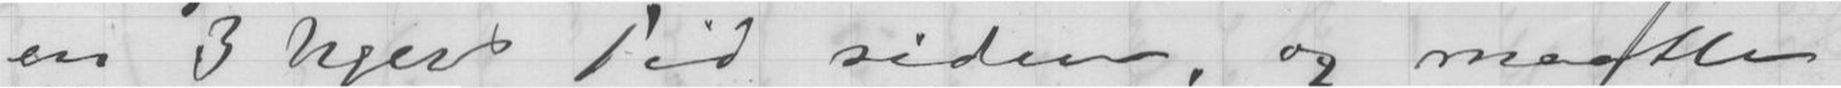en 3 Ugers Tid siden, og maatte
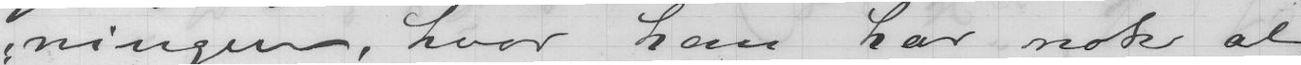ningen, hvor han har nok at
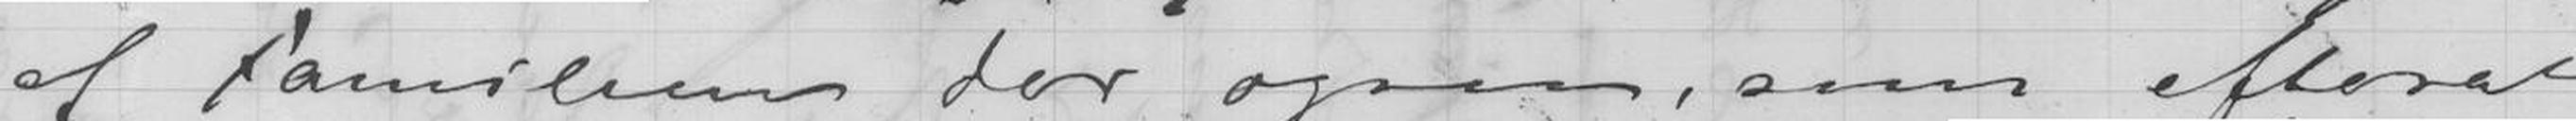af Familien der ogsaa, som efterat
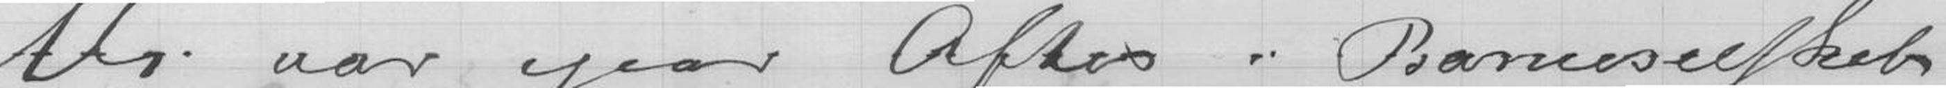Vi var igaar Aftes i Barneselskab
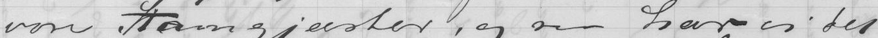vore Stamgjester, og saa har vi det
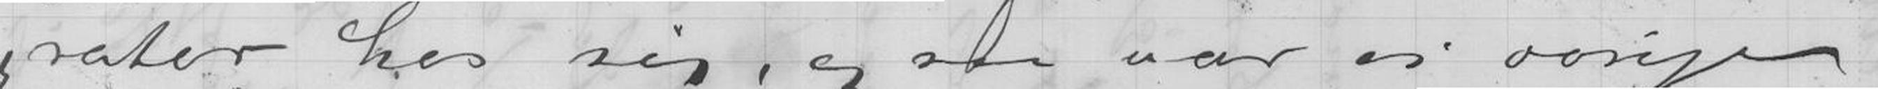rater hos sig, og saa var vi øvrige
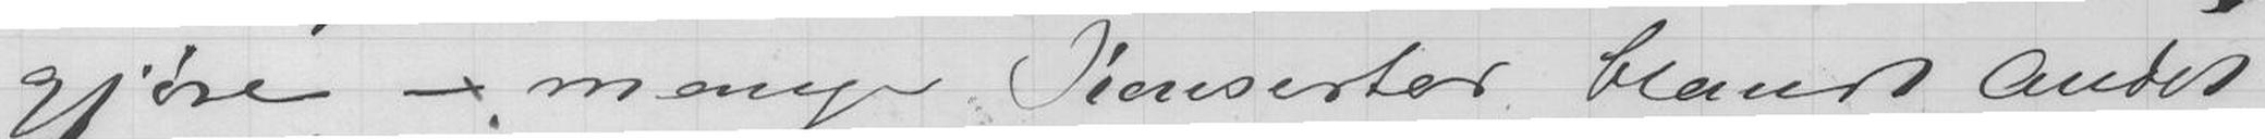gjøre - mange Konserter blandt Andet
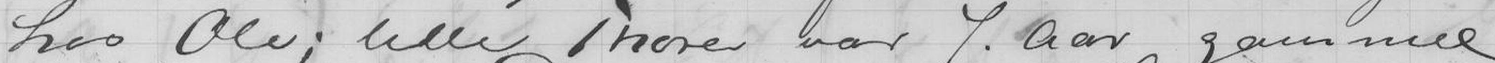hos Ole; lille Thora var 7 Aar gammel
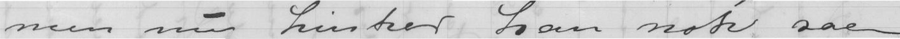men nu hinker han nok saa
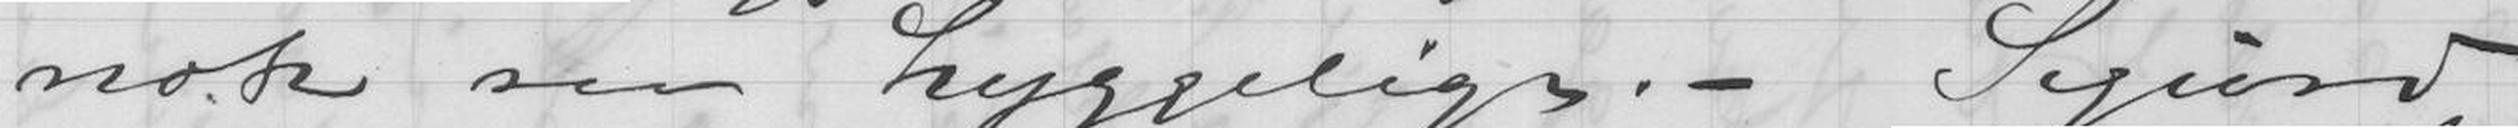nok saa hyggeligt. - Sigurd
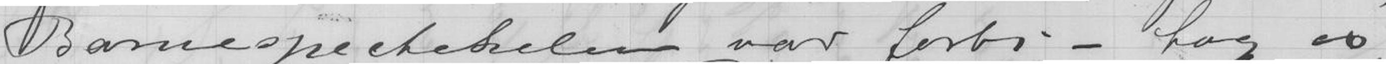Barnespeetakelen var forbi - tog os
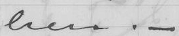lien. -
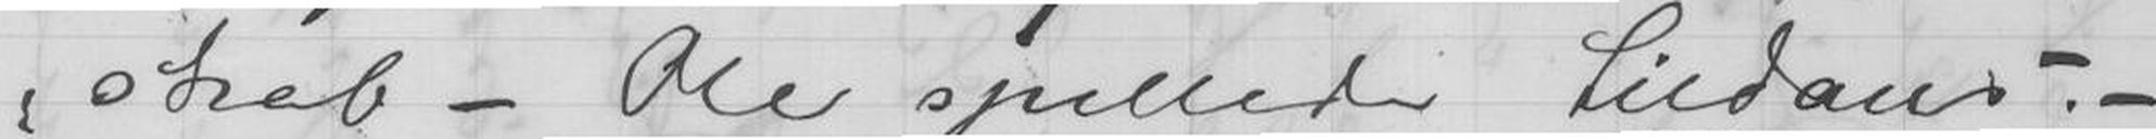skab - Ole spillede tildans. -
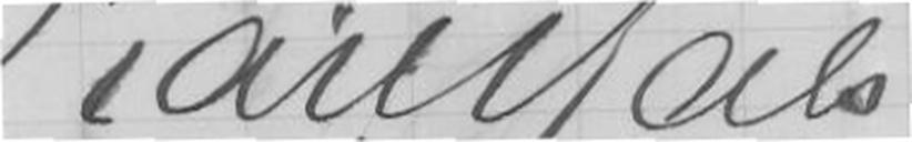Karl Hals
